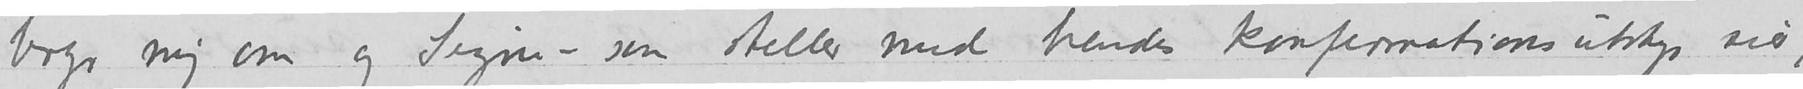bryr mig om og Signe – som steller med hendes konfirmationsutstyr sier,
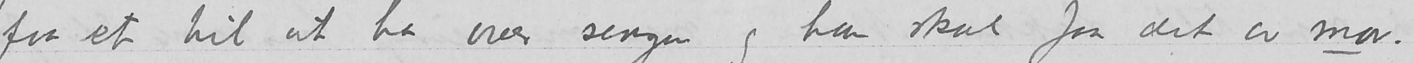faa et til at ha over sengen og han skal faa det av mor.
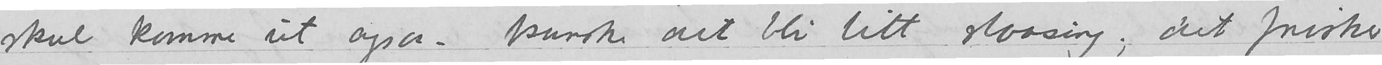skal komme ut ogsaa – kanske det blir litt slaasing; det frisker
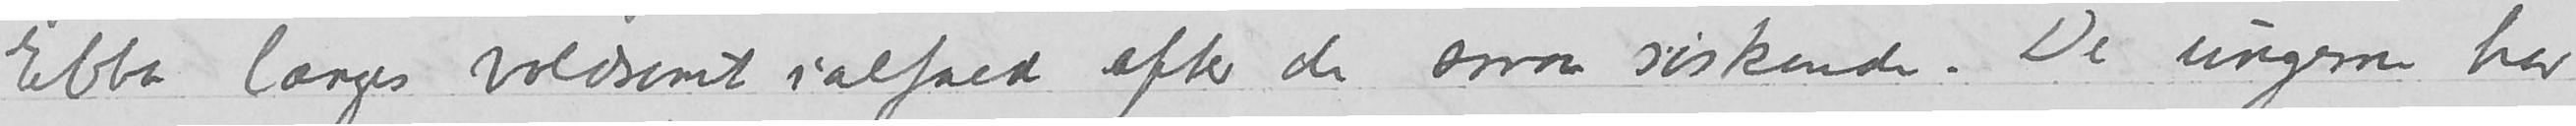Ebba længes voldsomt ialfald efter de smaa søskende. De ungerne har
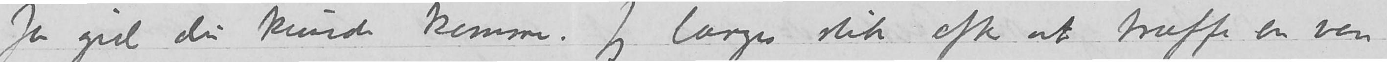Ja gid du kunde komme. Jeg længes slik efter at træffe en ven –
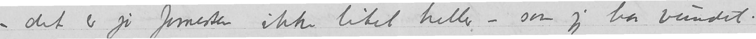det er jo forresten ikke litet heller – som jeg har vundet.
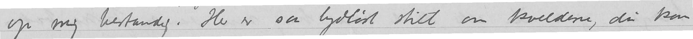op mig bestandig. Her er saa lydløst stilt om kvelderne, du kan
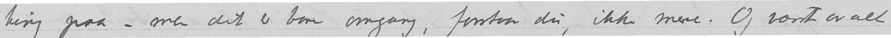ting paa – men det er bare omgang, forstaar du, ikke mere. Og værst av alt
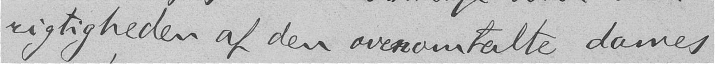rigtigheden af den overomtalte dames
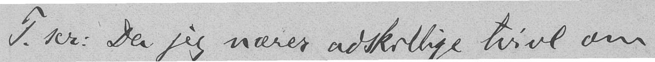P. scr: Da jeg nærer adskillige tvivl om
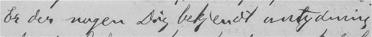Er der nogen Dig bekjendt antydning
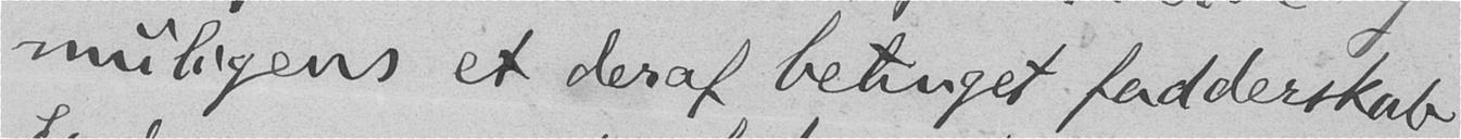muligens et deraf betinget fadderskab.
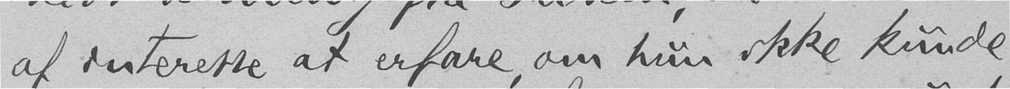af interesse at erfare, om hun ikke kunde
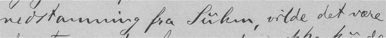nedstamning fra Suhm, vilde det være
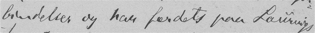bindelser og har færdets paa Laurvigs-
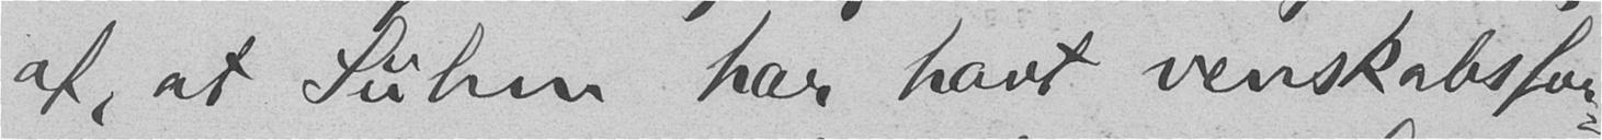af, at Suhm har havt venskabsfor-
